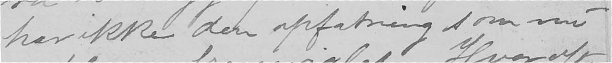har ikke den opfatning som nu
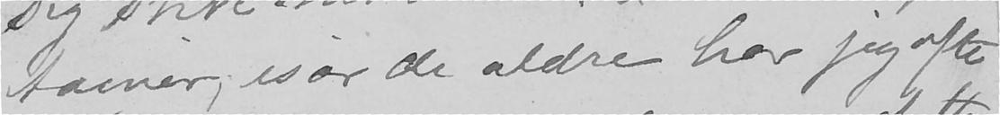teiner, især de ældre har jeg ofte
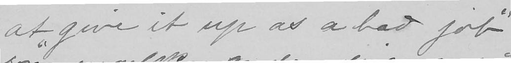at "give it up as a bad job"
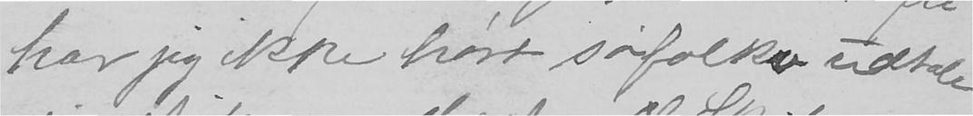har jeg ikke hørt søfolker udtale
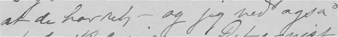at de har ret, - og jeg ved også
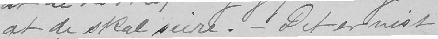at de skal seire. - Det er vist
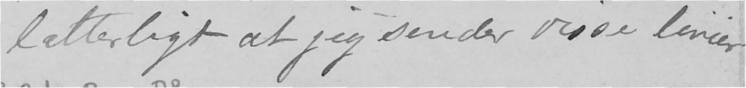latterligt at jeg sender disse linier
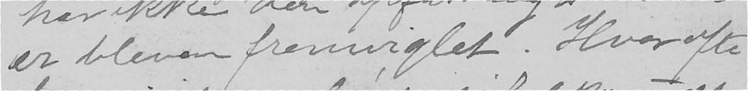er bleven fremviglet. Hvor ofte
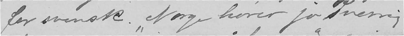for svensk. "Norge hører jo Sverrig
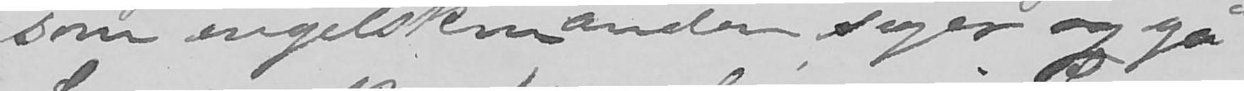som engelskemanden siger og gå
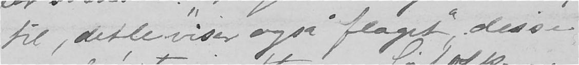til, dette viser også flaget", disse
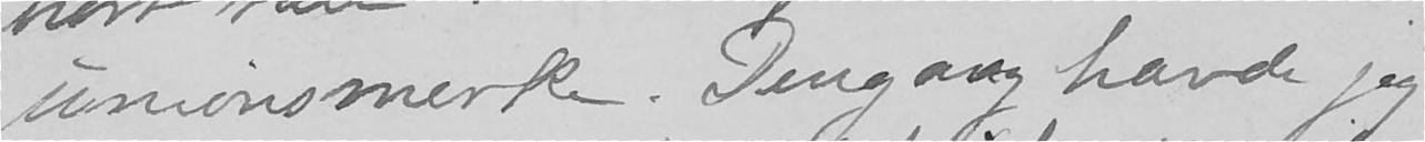unionsmerke. Dengang havde jeg
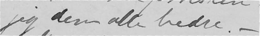jeg dem alle bedre. -
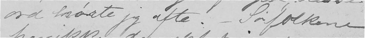ord hørte jeg ofte. Søfolkene
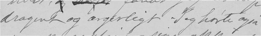dragent og ærgerligt. Jeg hørte også
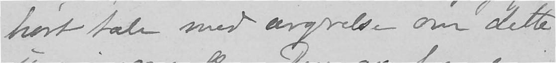hørt tale med ærgrelse om dette
